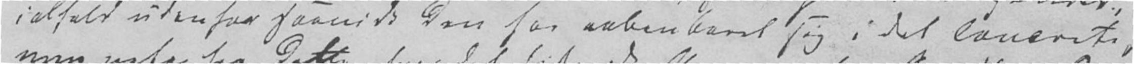ialfald udenfor saavidt den har aabenbaret sig i det Concrete,
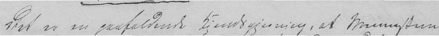Det er en paafaldende Kjendsgjerning, at Menneskene
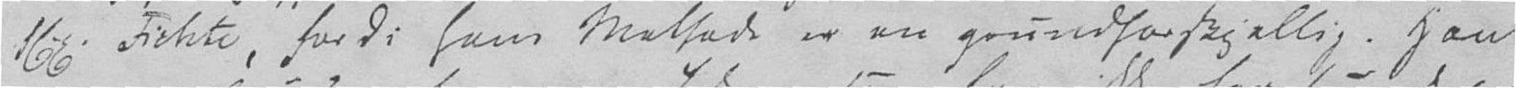fEx. Fichte, fordi hans Methode er en grundforskjællig. Han
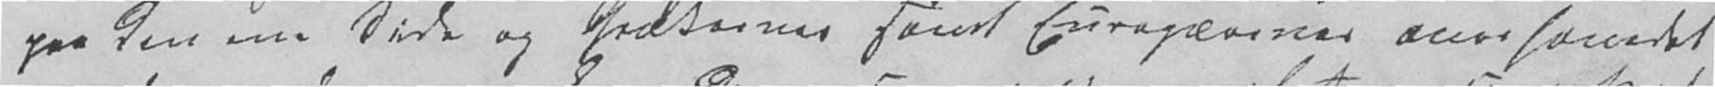paa den ene Side og Grækernes samt Europeernes overhovedet
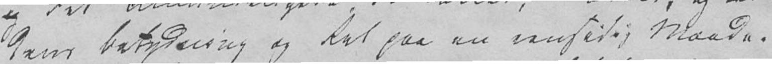dens Betydning og Ret paa en eensidig Maade.
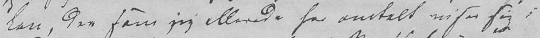Lov, der som jeg allerede har omtalt viser sig i
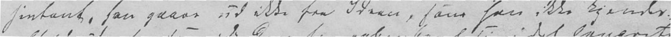sentant, som gaaer ud ikke fra Ideen, som han ikke kjender,
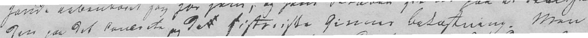den paa det concrete og det historiske Givnes Bekostning. Men
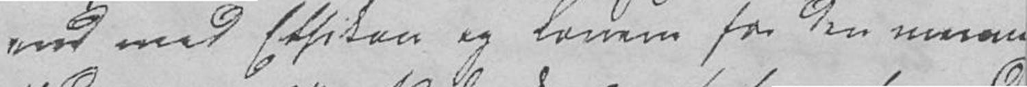end med Ethiken og Lovene for den menne-
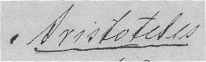Aristoteles
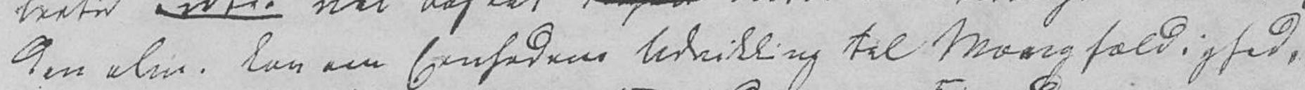den alm. Lov om Eenhedens Udvikling til Mangfoldighed,
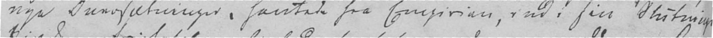nye Oversætninger, hentede fra Empirien, ind i sin Slutnings-
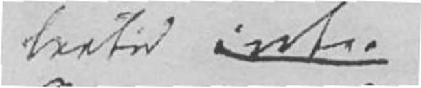lertid X
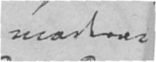moderne
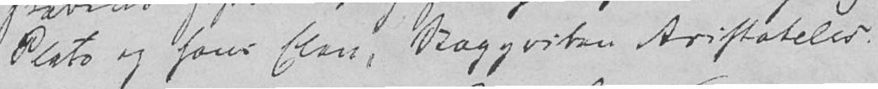Plato og hans Elev, Stagyriten Aristoteles.
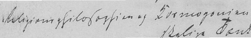Religionsphilosophien og Kosmogonien
